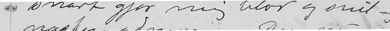snart gjør mig blød og snil og
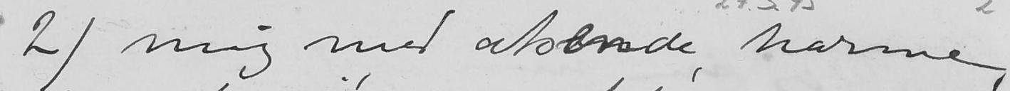2) mig med ætsende harme,
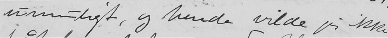umuligt, og hende vilde jeg ikke
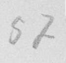57
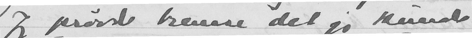Jeg prøvde bremse det jeg kunde
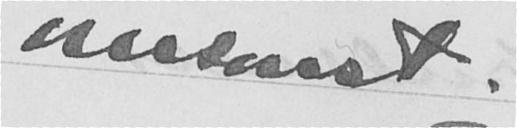indsast
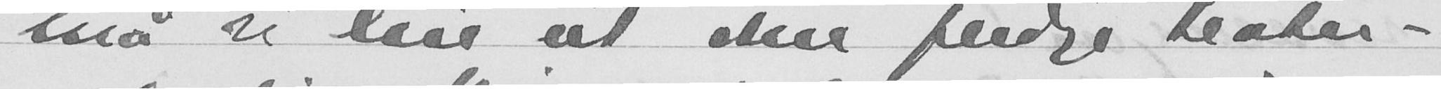må vi leie ut den ferdige teater-
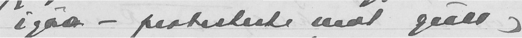igår - protesterte mot gult og
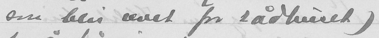om blir revet for rådhuset)
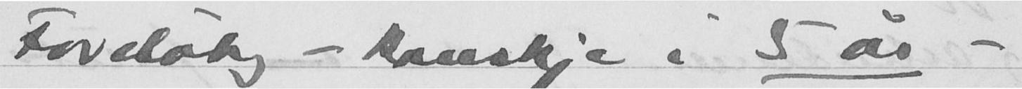Foreløbig - kanskje i 5 år -
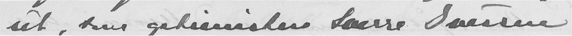ut, som optimisten Sverre Svenson
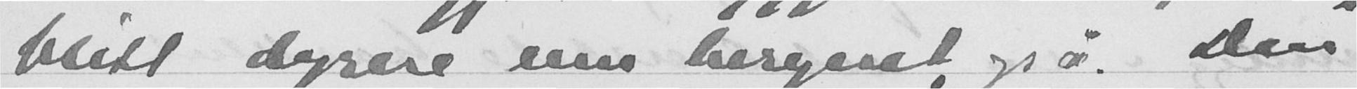blitt dyrere enn beregnet også. Den
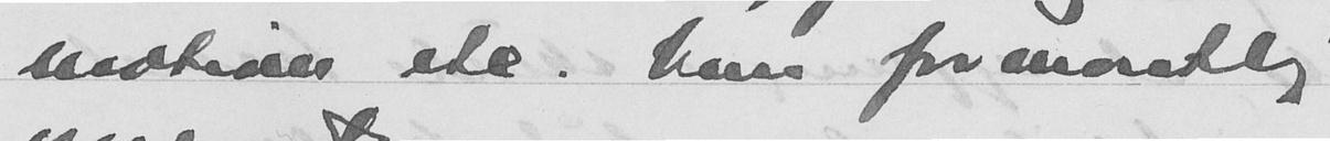motiver etc. Kun formastlig
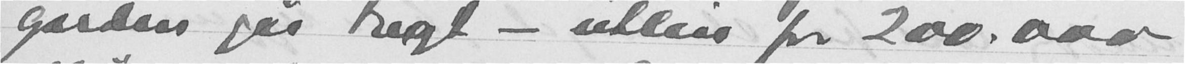gården går tregt - utleie for 200.000
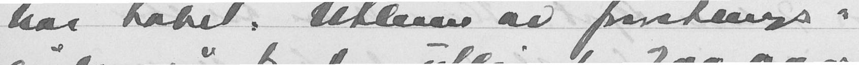har håbet. Utleien av foretnings-
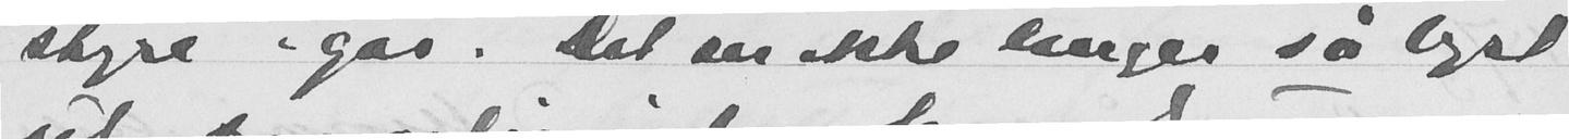styre i går. Det ser ikke lenger så lyst
